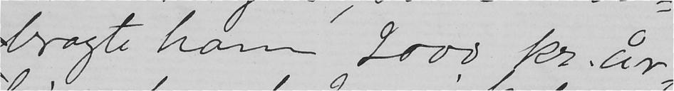bragte ham 2000 kr. år-
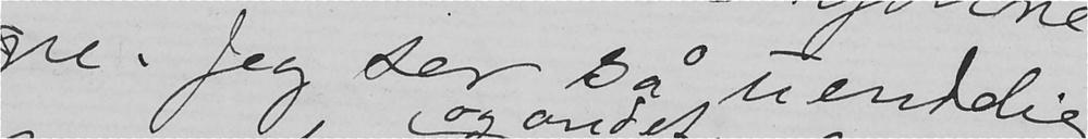ne. Jeg ser så uendelig
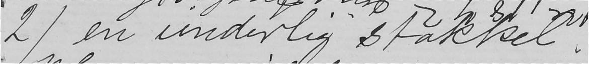2) en underlig stakkel.
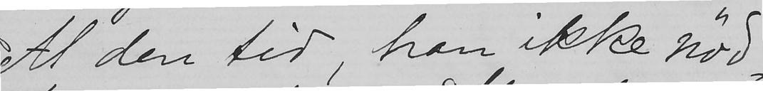Al den tid, han ikke nød-
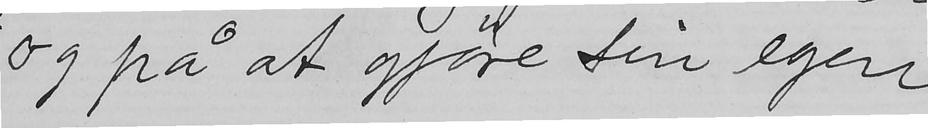og på at gjøre sin egen
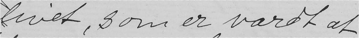livet, som er værdt at
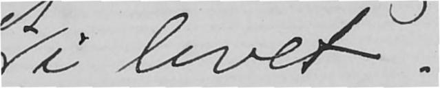i livet.
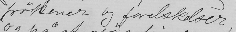frøkener og forelskelser,
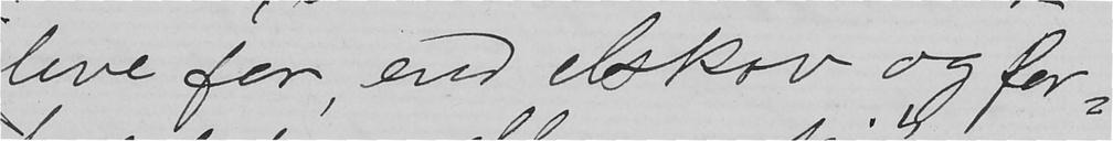leve for, end elskov og for-
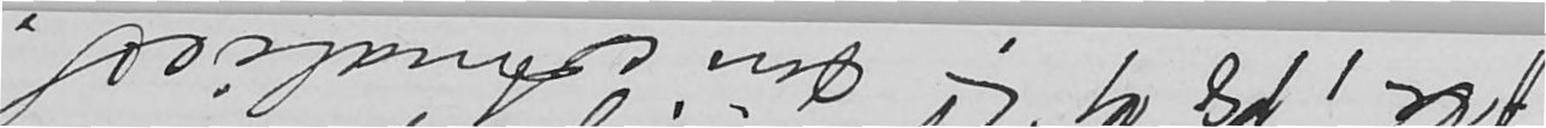pe, 187? Din Amalie S.
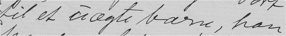til et uægte barn, han
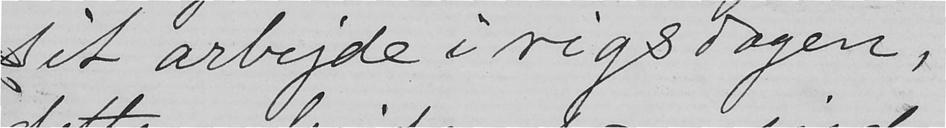Det arbejde i rigsdagen,
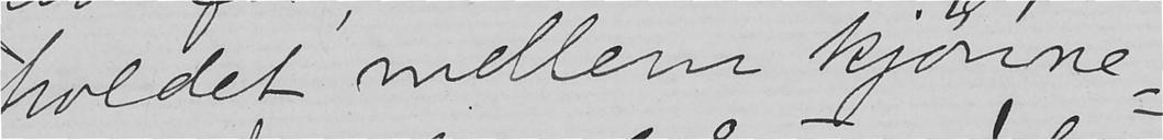holdet mellem kjønne-
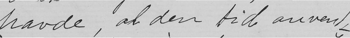havde, al den tid anvendte
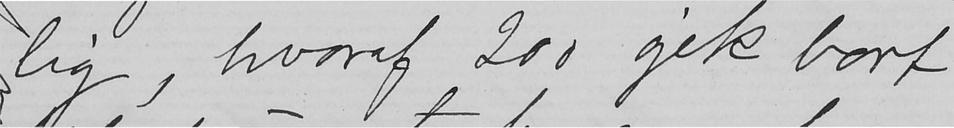lig, hvoraf 200 gik bort
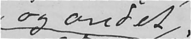og andet
